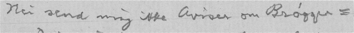Nei send mig ikke Aviser om Brøgger-
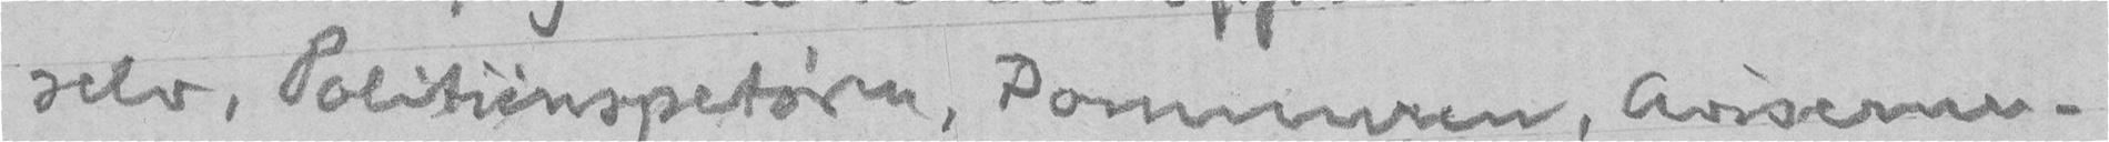selv, Politiinspektøren, Dommeren, Aviserne.
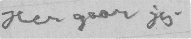Her gaar jeg.
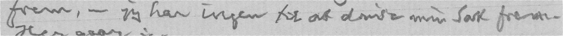frem, - jeg har ingen til at drive min Sak frem.
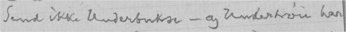Send ikke Underbukse - og Undertrøie har
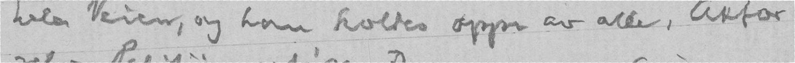hele Veien, og han holdes oppe av alle, Aktor
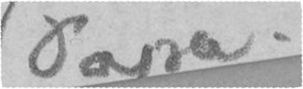Papa.
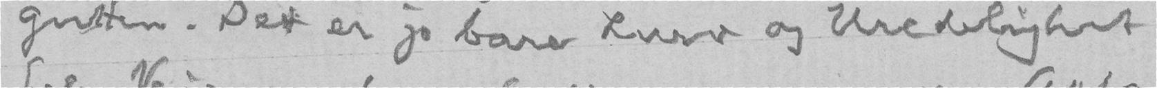gutten. Det er jo bare Lurv og Uredelighet
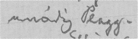unødig Plagg.
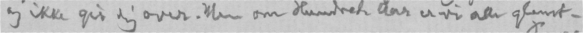og ikke gir dig over. Men om Hundrede Aar er vi alle glemt.
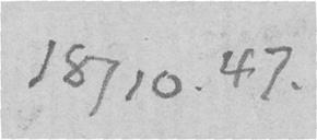18/10. 47.
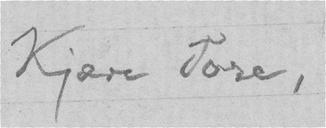Kjære Tore,
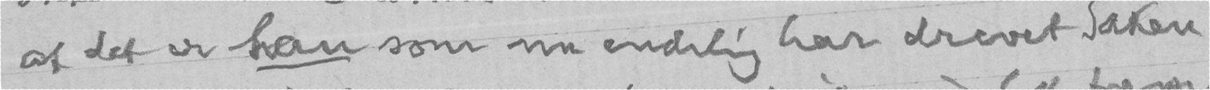at det er han som nu endelig har drevet Saken
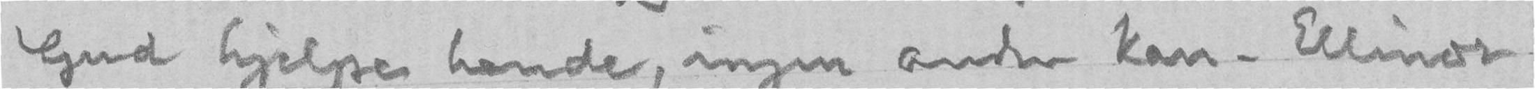Gud hjelpe hende, ingen anden kan. Ellinor
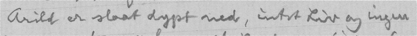Arild er slaat dypt ned, intet Liv og ingen
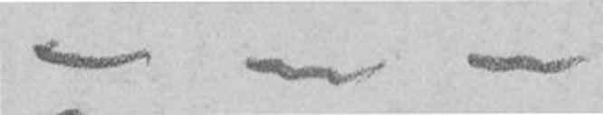- - -
